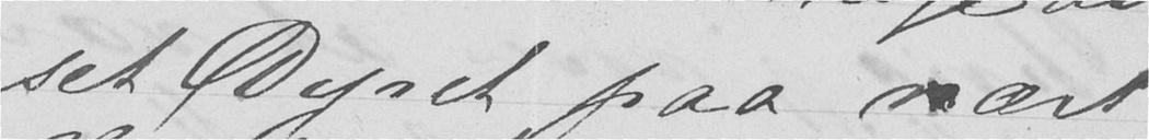set Dyret paa nært
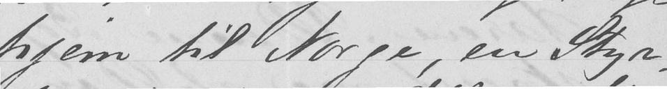hjem til Norge, en Styr-
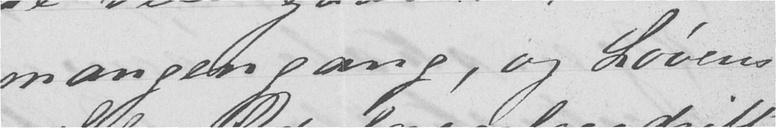mangen gang, og Løvens
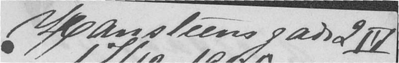Hansteens gade 2 IV
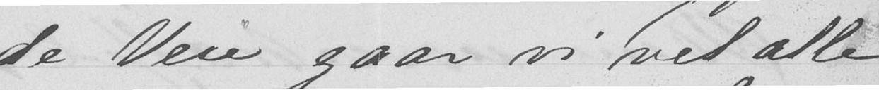de Veie gaar vi vel alle
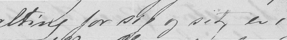alting for sig og sit, er i
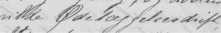vilde Ødelæggelsesdrift,
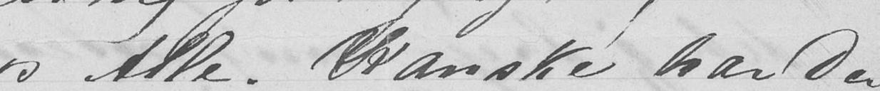os Alle. Kanske har Du
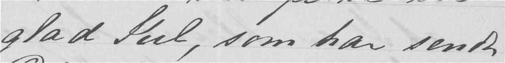glad Jul, som har sendt
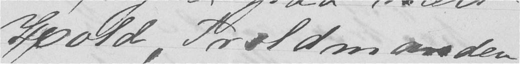Hold, Troldmanden,
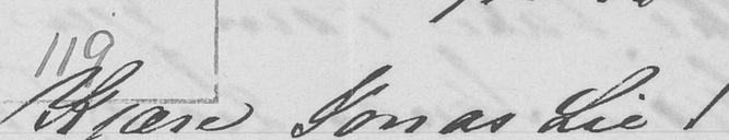Kjære Jonas Lie!
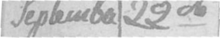Septemer 22de
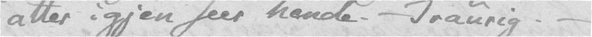atter igjen seer hende. – Traurig. –
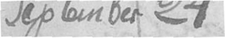September 24
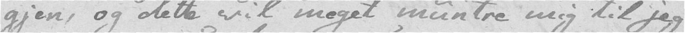gjen, og dette vil meget muntre mig til jeg
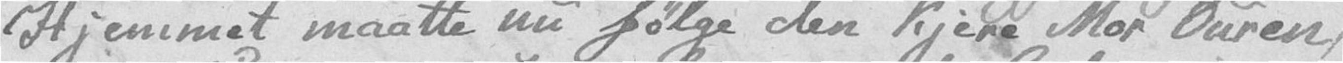Hjemmet maatte nu følge den kjere Mor Ouren,
